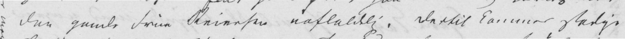den gamle Frue Reiersen uafladelig. Dertil kommer stadige
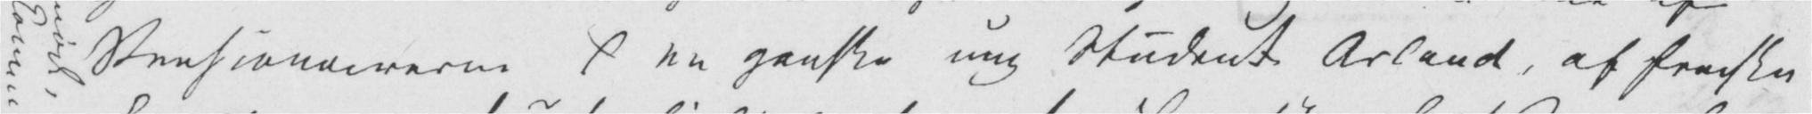Pensionairerne ( en ganske ung Student Arland, af franske
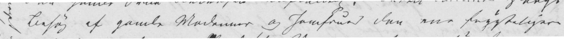Besøg af gamle Madammer og Jomfruer den ene frygteligere
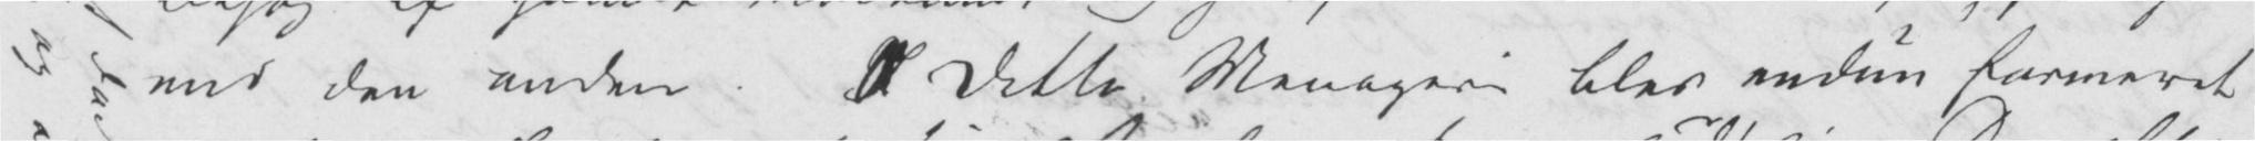end den anden. Dette Menagerie blev endnu formeret
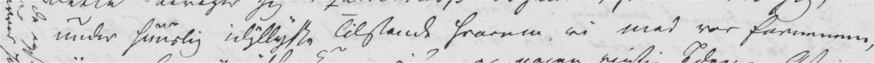under huuslig idylliske Tilstande hvorom vi med vor fornemme,
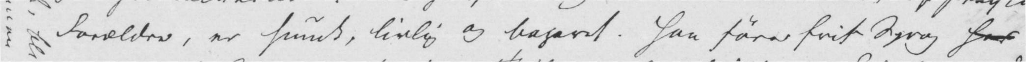Forældre, er smuk, livlig og begavet. Han fører frit Sprog her
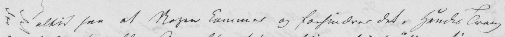altid saa at Nogen kommer og forhindrer det, Hendes Trang
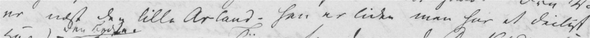er næst den lille Arland – han er liden men har et deiligt
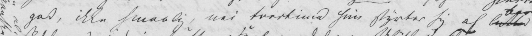god, ikke smaalig, nei tvertimod hun styrter sig af lutter
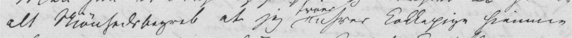alt Skjønhedsbegreb at jeg enhver Kokkepige hjemme
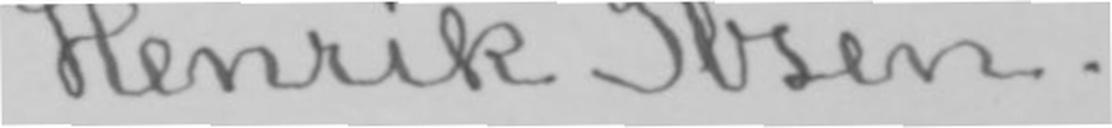Henrik Ibsen.
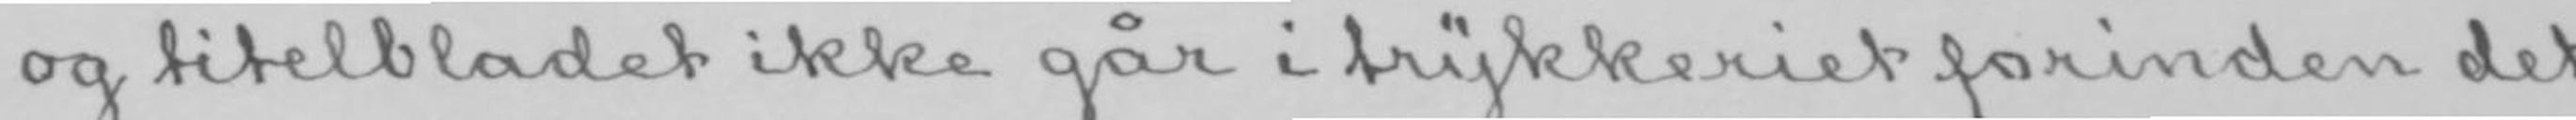og titelbladet ikke går i trykkeriet forinden det
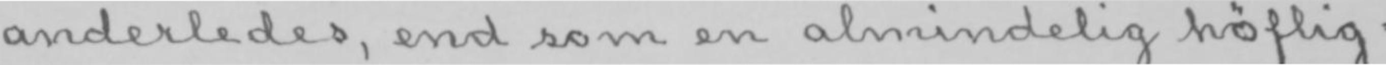anderledes, end som en almindelig høflig-
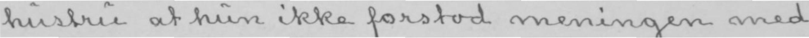hustru at hun ikke forstod meningen med
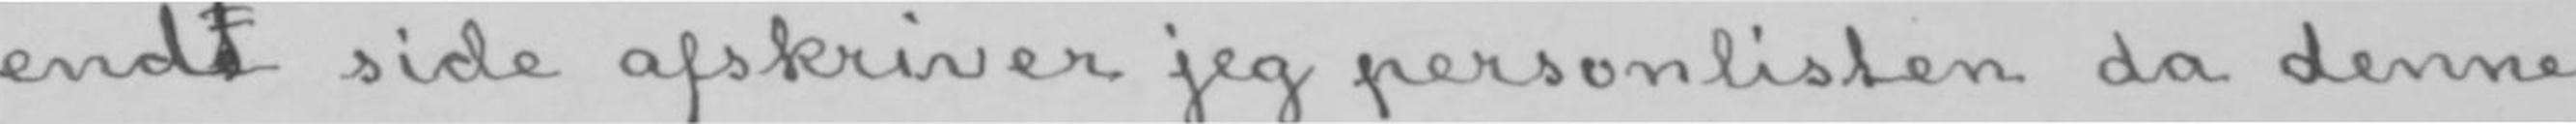endte side afskriver jeg personlisten da denne
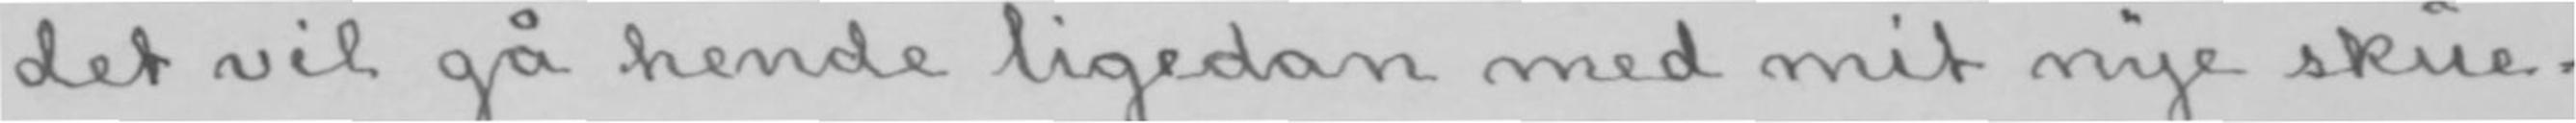det vil gå hende ligedan med mit nye skue-
